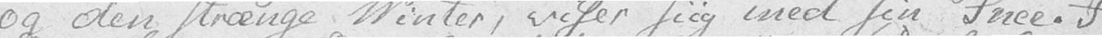og den strænge Vinter, viser siig med sin Snee. I
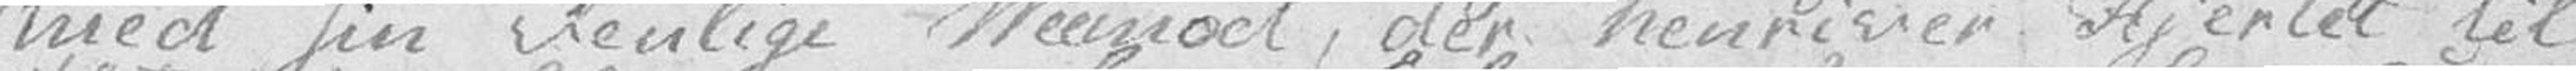med sin venlige Veemod, der henriver Hjertet til
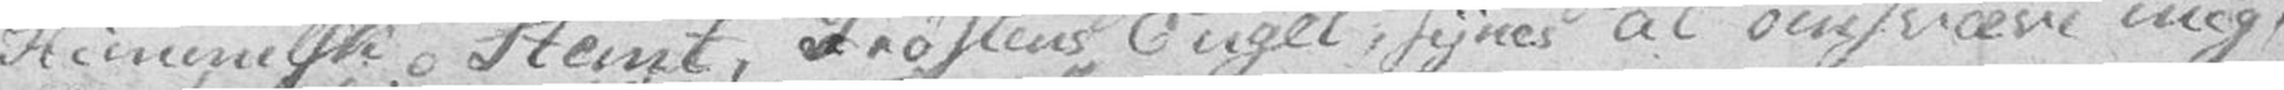Himmelsk Stemt, Trøstens Engel, synes at omsvæve mig,
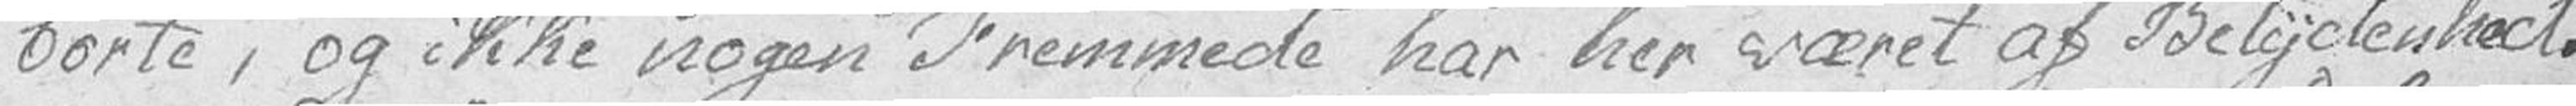borte, og ikke nogen Fremmede har her været af Betydenhed.
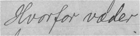Hvorfor væder
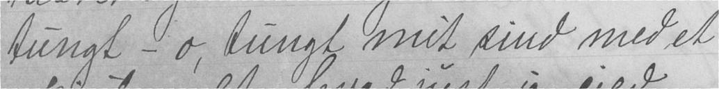tungt - o, tungt mit sind med et
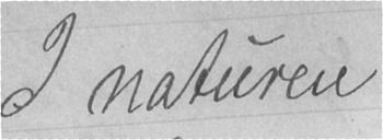I naturen
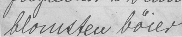blomsten bøier
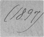(1897)
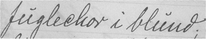fuglechor i blund;
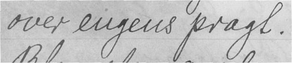over engens pragt.
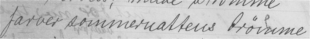farver sommernattens drømme
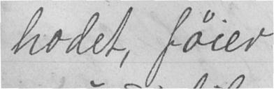hodet, føier
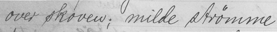over skoven; milde strømme
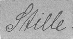Stille.
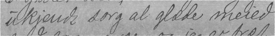ukjendt sorg al glæde meied
